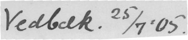Vedbæk. 25/7'05.
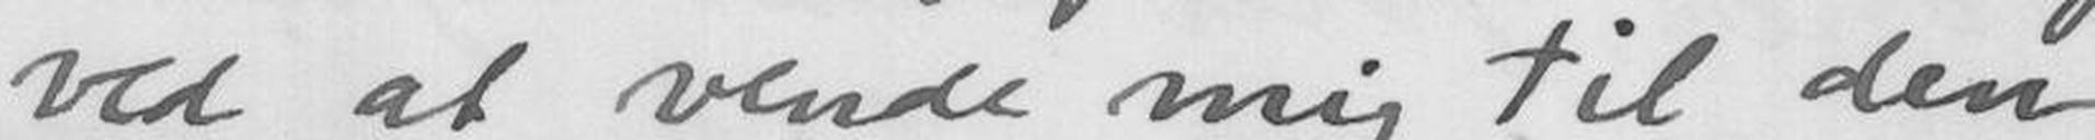ved at vende mig til den
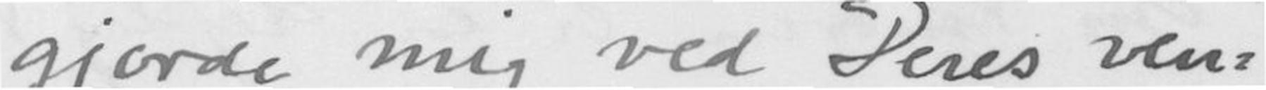gjorde mig ved Deres ven-
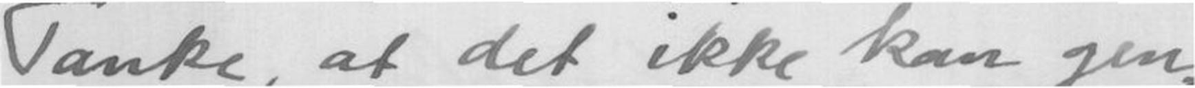Tanke, at det ikke kan gen-
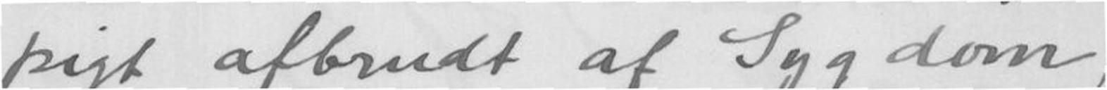pigt afbrudt af Sygdom,
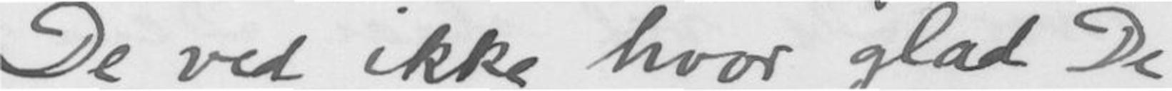De ved ikke hvor glad De
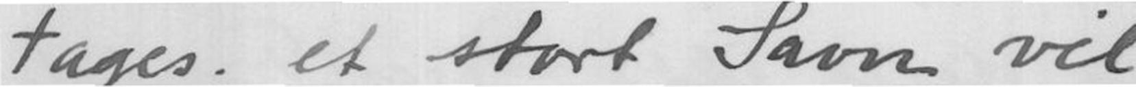tages; et stort Savn vil
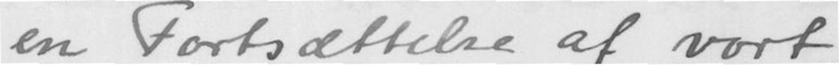en Fortsættelse af vort
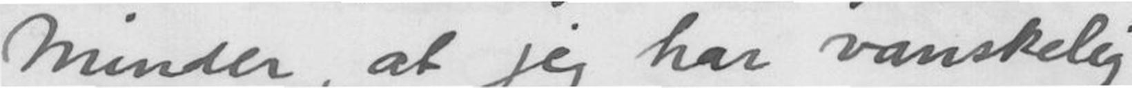Minder, at jeg har vanskelig
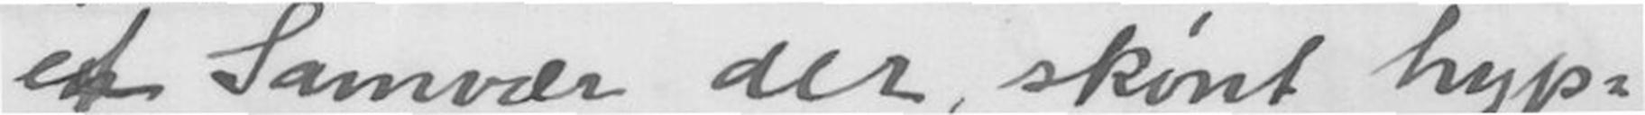et Samvær der, skønt hyp-
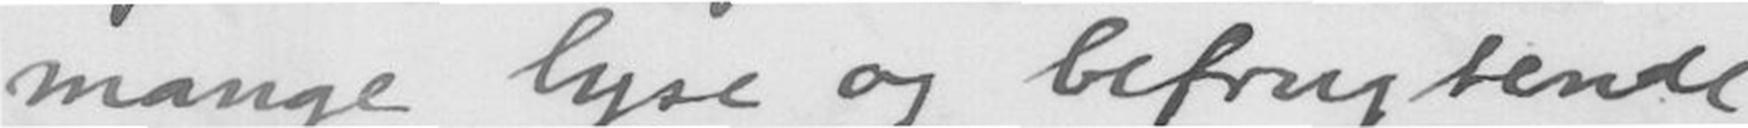mange lyse og befrugtende
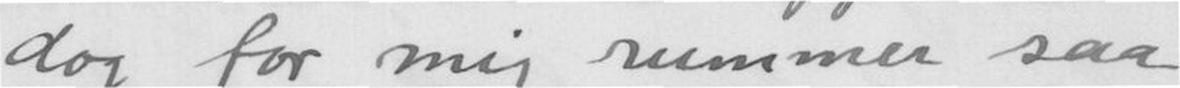dog for mig rummer saa
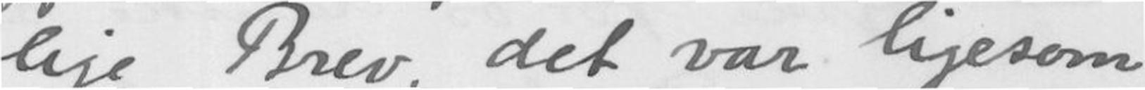lige Brev, det var ligesom
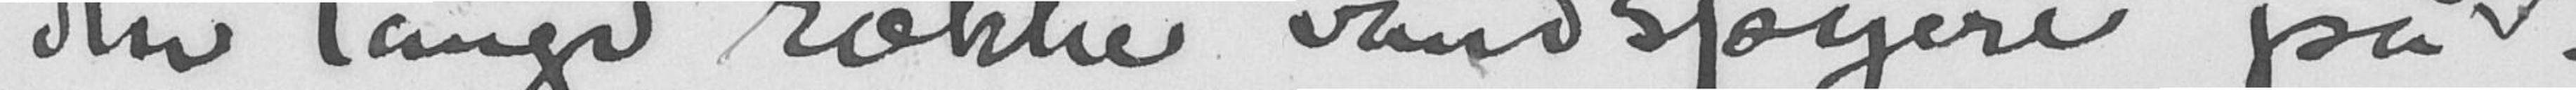den lange række vandspyere på
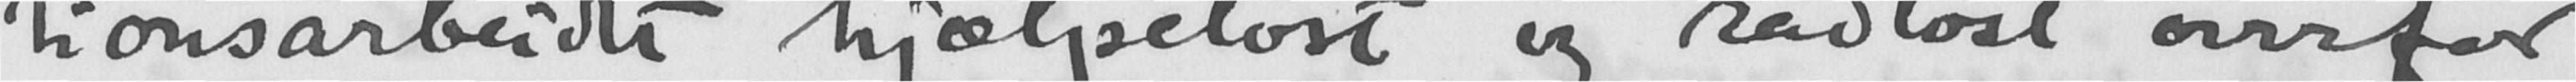tionsarbeidet hjælpeløst og rådløst overfor
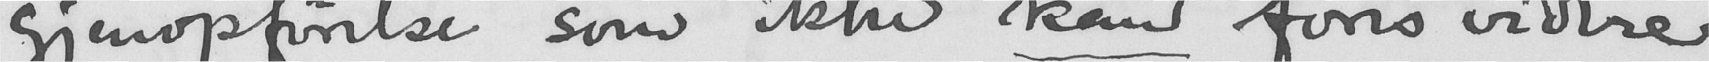gjenopførelse som ikke kan føres vidre
